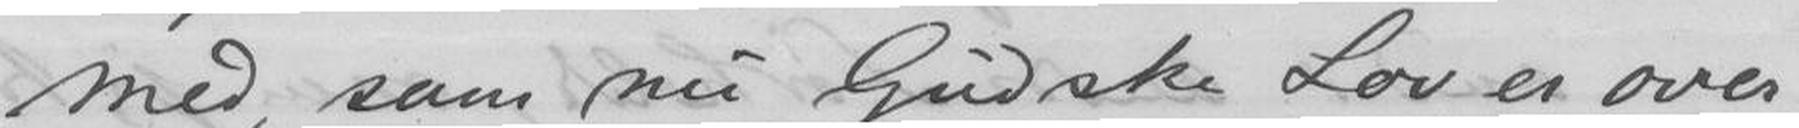med, som nu Gudske Lov er over,
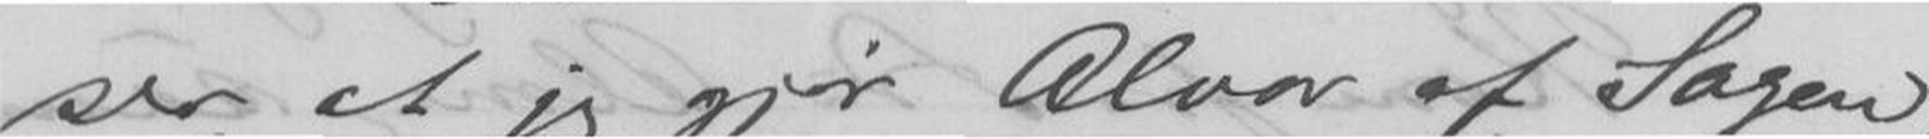ser at jeg gjør Alvor af Sagen,
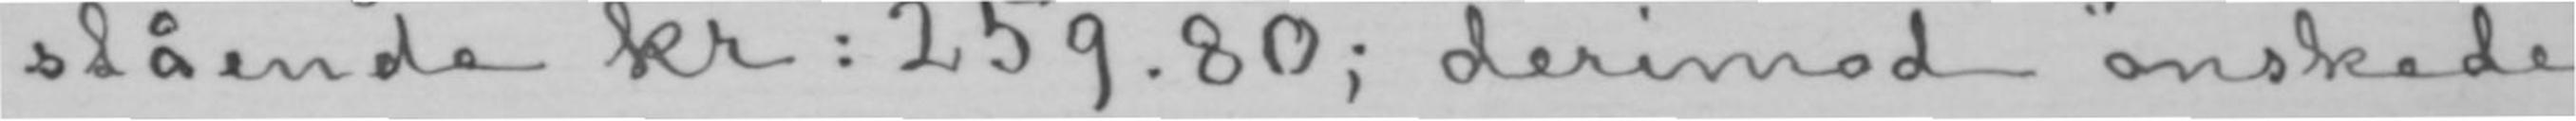stående kr: 259.80; derimod ønskede
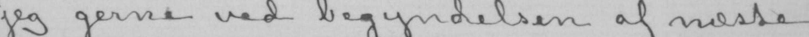jeg gerne ved begyndelsen af næste
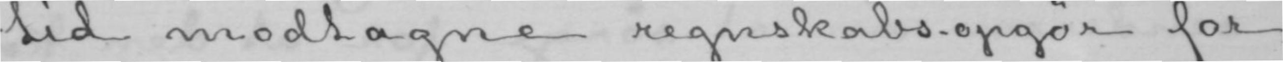tid modtagne regnskabs-opgør for
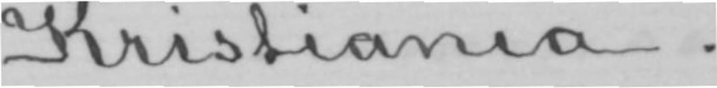Kristiania.
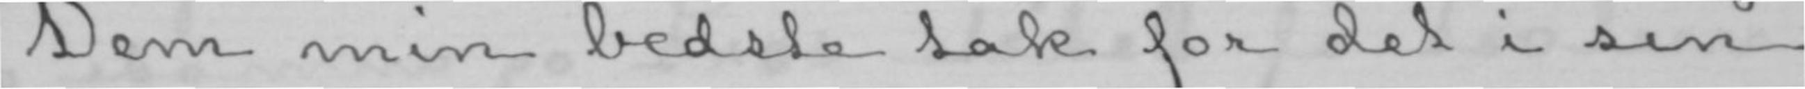Dem min bedste tak for det i sin
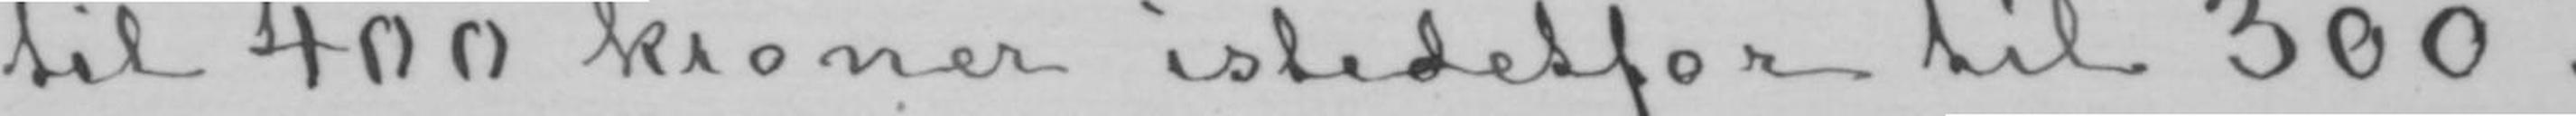til 400 kroner istedetfor til 300.
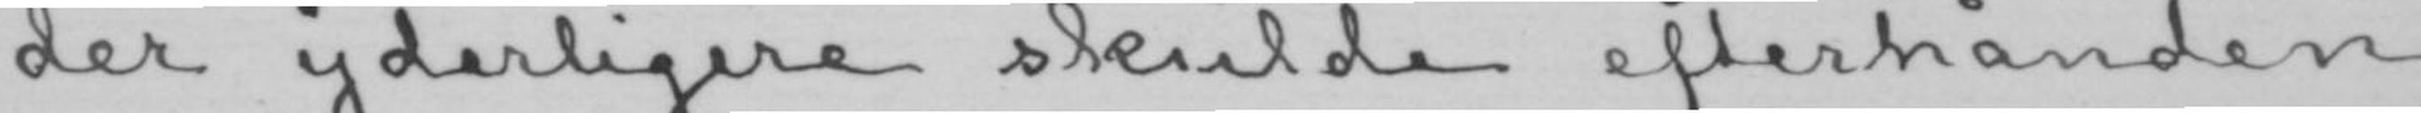der yderligere skulde efterhånden
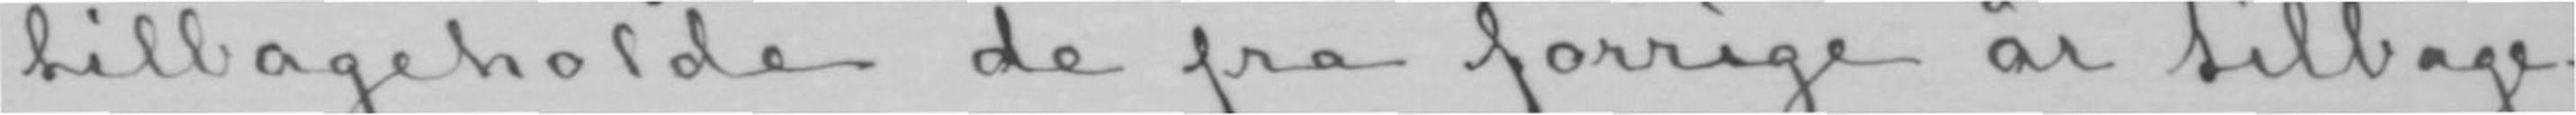tilbageholde de fra forrige år tilbage-
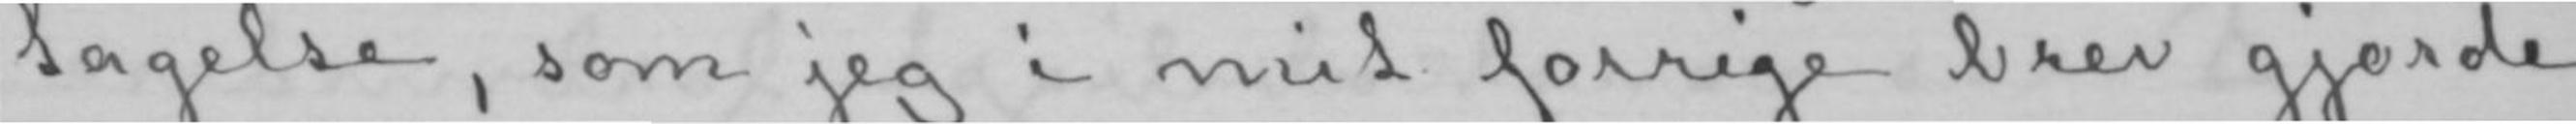tagelse, som jeg i mit forrige brev gjorde
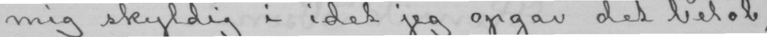mig skyldig i idet jeg opgav det beløb,
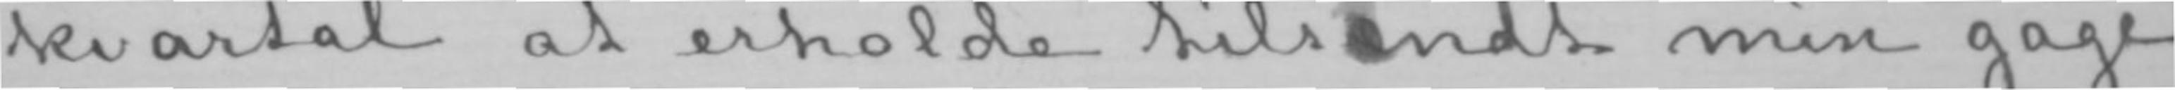kvartal at erholde tilsendt min gage
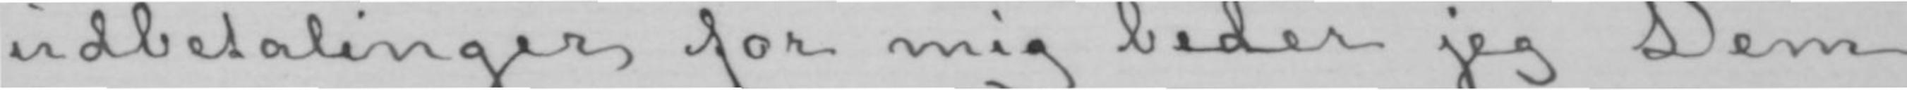udbetalinger for mig beder jeg Dem
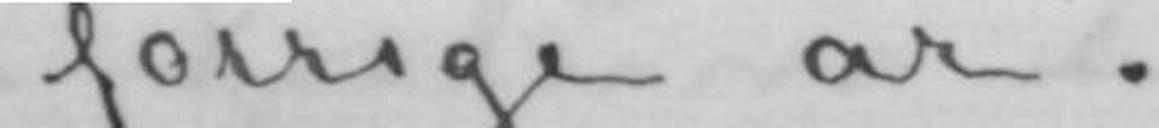forrige år.
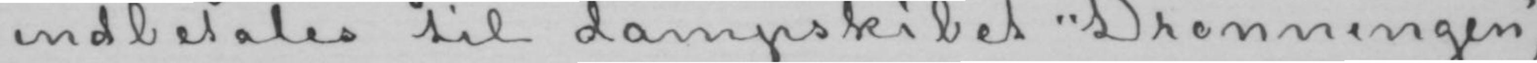indbetales til dampskibet «Dronningen»,
